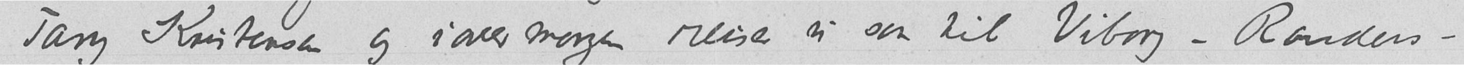Tang Kristensen og iovermorgen reiser vi saa til Viborg – Randers –
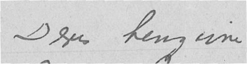Deres hengivne
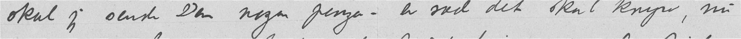skal jeg sende Dem nogen penger – er ræd det skal knipe, nu
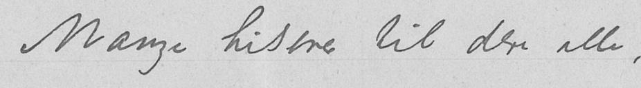Mange hilsener til dere alle,
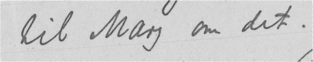til Mary om det.
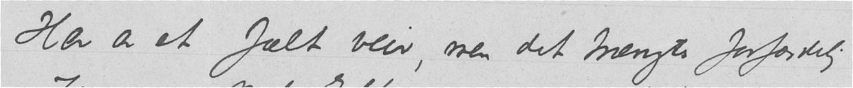Her er et fælt veir, men det trængtes forfærdelig
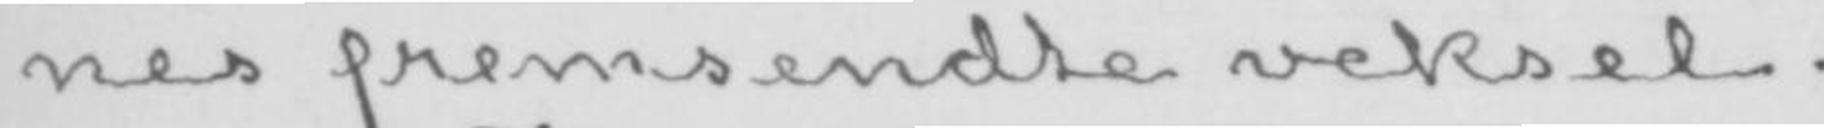nes fremsendte veksel.
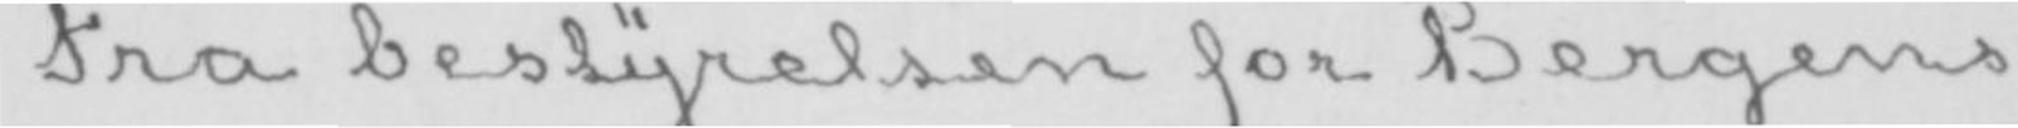Fra bestyrelsen for Bergens
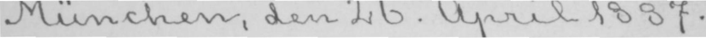München, den 26. April 1887.
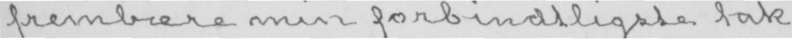frembære min forbindtligste tak
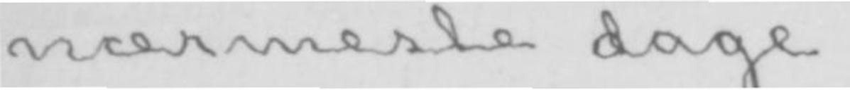nærmeste dage.
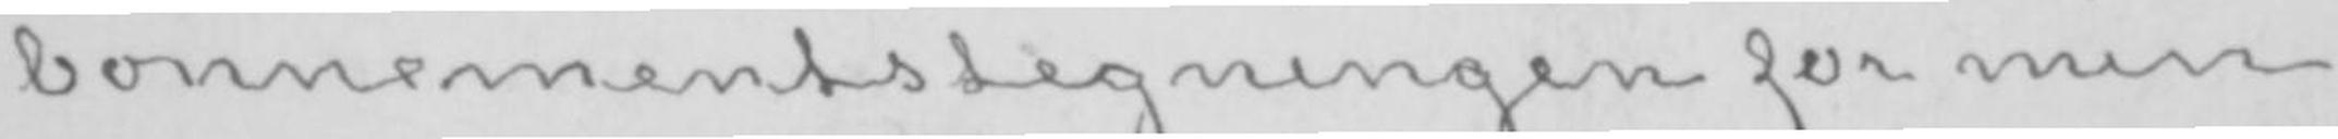bonnementstegningen for min
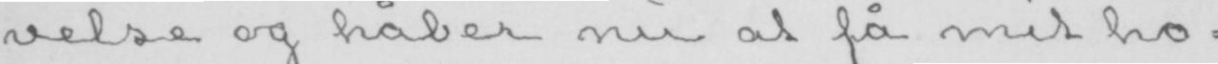velse og håber nu at få mit ho-
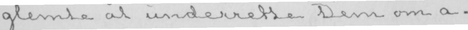glemte at underrette Dem om a-
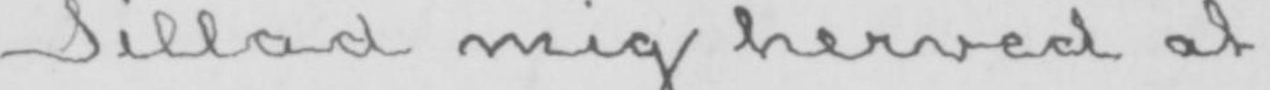Tillad mig herved at
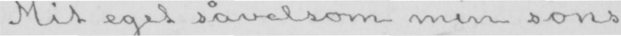Mit eget såvelsom min søns
